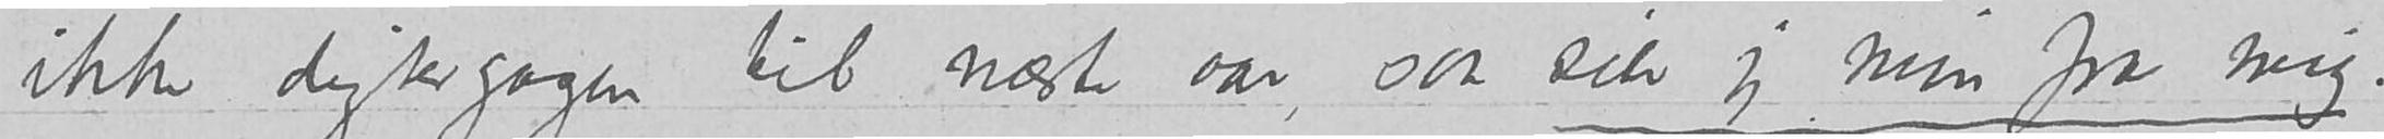ikke digtergagen til næste aar, saa sier jeg min fra mig.
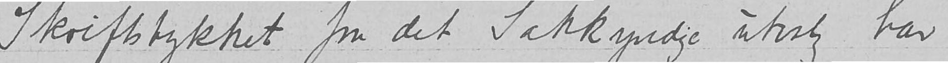Skriftstykket fra det Sakkyndige utvalg har
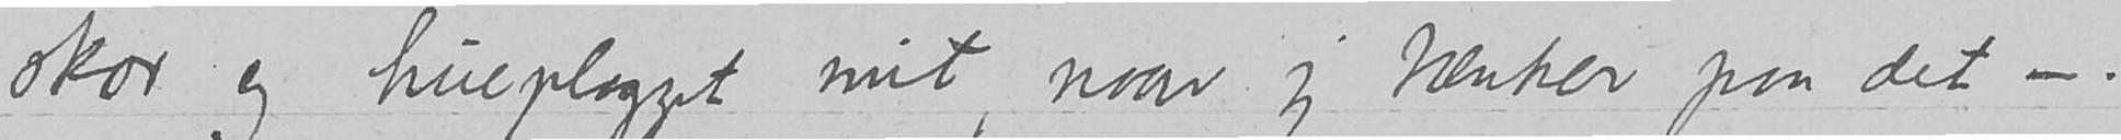skor og hueplagget mit, naar jeg tænker paa det -
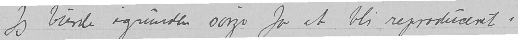Jeg burde igrunden sørge for at bli reproduceret i
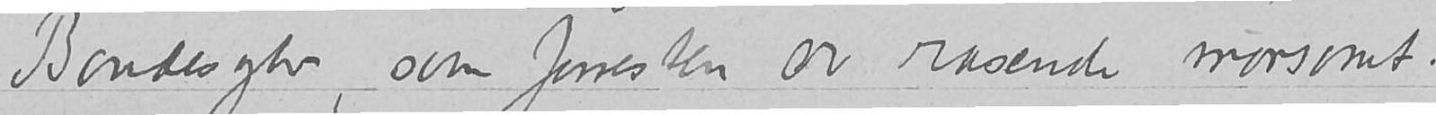Bondesylv, som forresten er rasende morsomt.
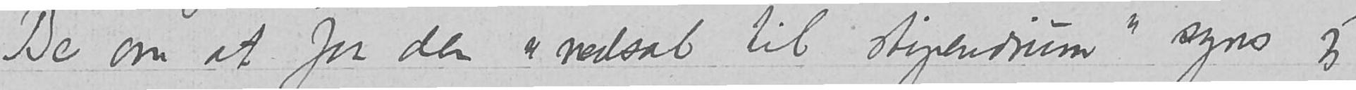Be om at faa den «nedsat til stipendium» syns jeg
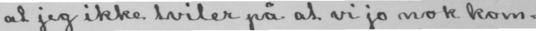at jeg ikke tviler på at vi jo nok kom-
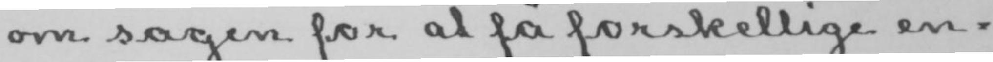om sagen for at få forskellige en-
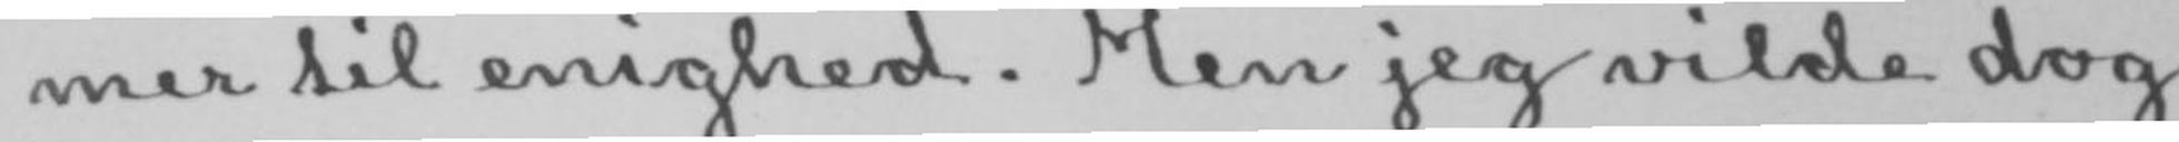mer til enighed. Men jeg vilde dog
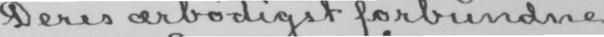Deres ærbødigst forbundne
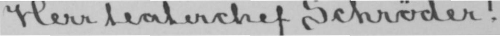Herr teaterchef Schrøder!
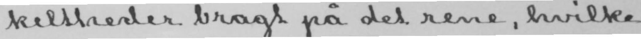keltheder bragt på det rene, hvilke
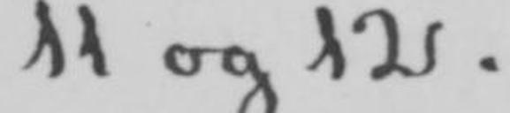11 og 12.
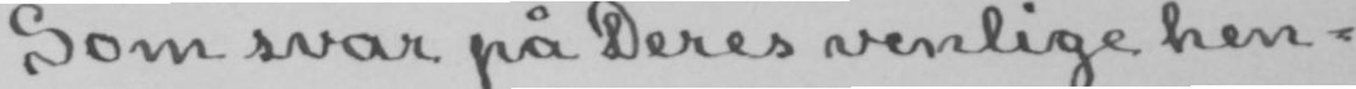Som svar på Deres venlige hen-
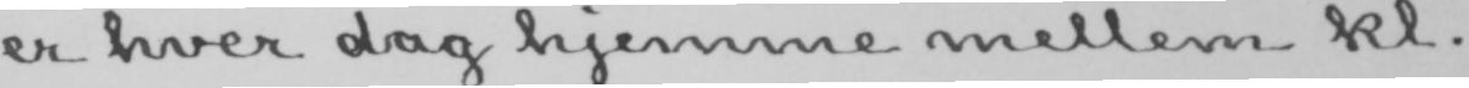er hver dag hjemme mellem kl.
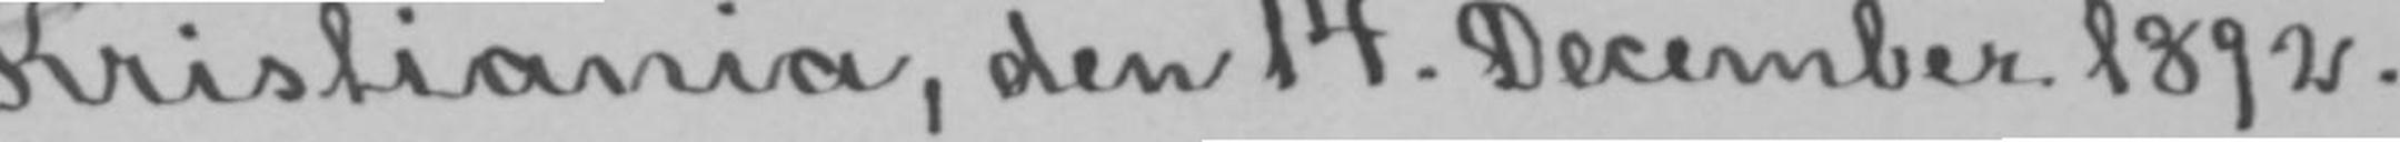Kristiania, den 14. December 1892.
